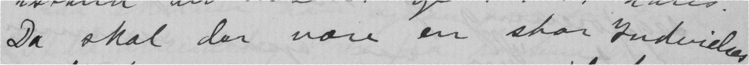Da skal der være en stor Indvilses
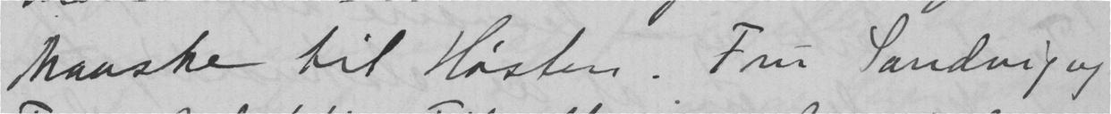Maaske til Høsten. Fru Sandvig og
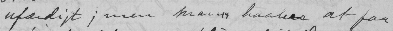ufærdigt; men man " haaber at faa
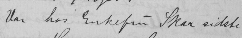Var hos Enkefru Skar sidste
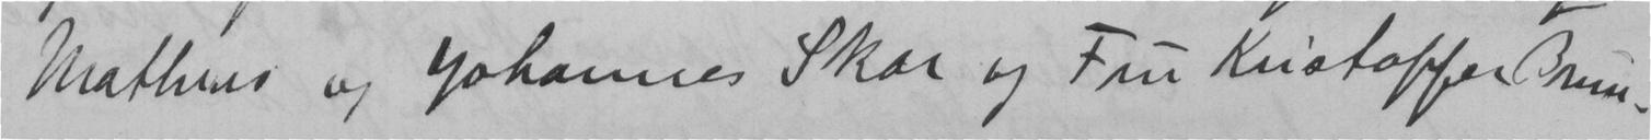Mathus og Johannes Skar og Fru Kristoffer Brun.
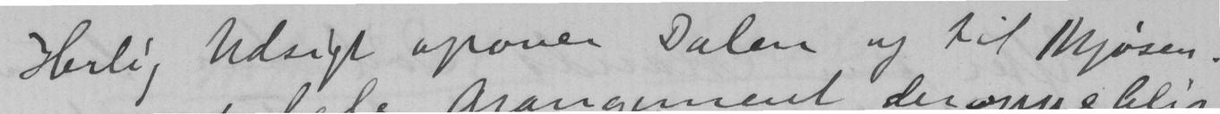Herlig Udsigt opover Dalen og til Mjøsen.
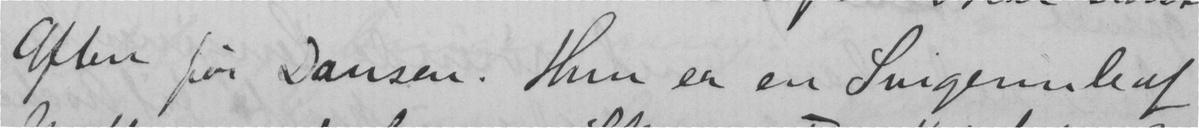Aften før Dansen. Hun er en Svigerinde af
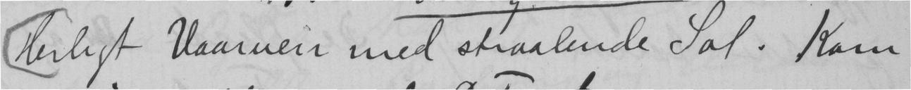Herligt Vaarveir med straalende Sol. Kom
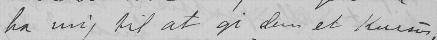ha mig til at gi dem et Kursus,
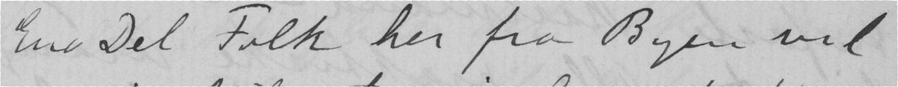En Del Folk her fra Byen vil
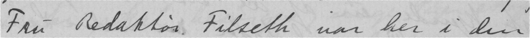Fru Redaktør Filseth var her i den
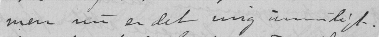men nu er det mig umuligt.
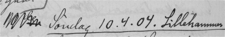Sønlag 10.4.04. Lillehammer
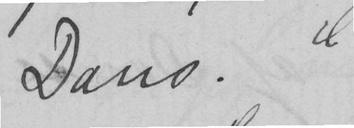Dans. "
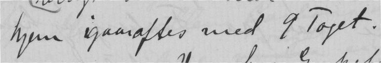hjem iaaraftes med 9 Toget.
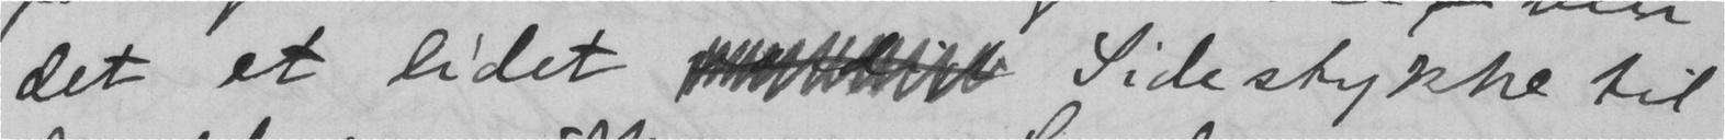det et lidet  Sidestykke til
